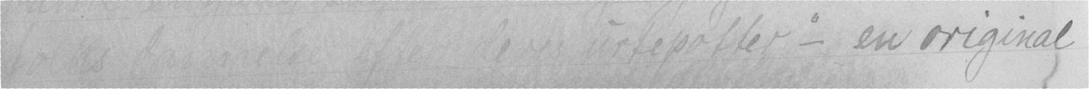folks dannelse efter deres urtepotter" - en original
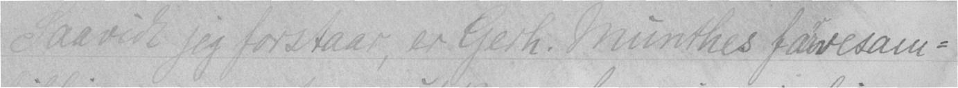Saavidt jeg forstaar, er Gerh. Munthes farvesam-
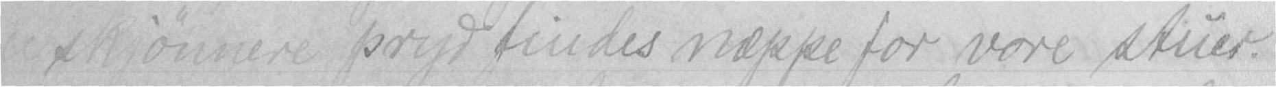en skjønnere pryd findes næppe for vore stuer.
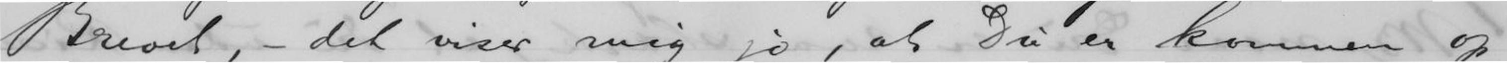Brevet, - det viser mig jo, at Du er kommen op
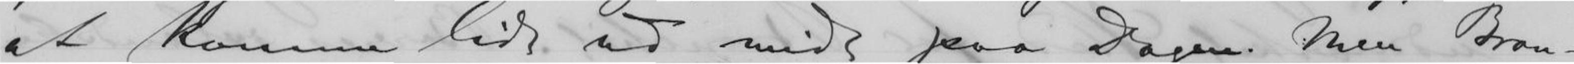at komme lidt ud midt paa Dagen. Men Bron-
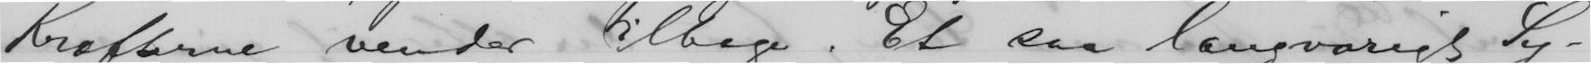Kræfterne vender tilbage. Et saa langvarigt Sy-
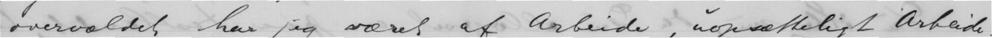overvældet har jeg været af Arbeide, uopsætteligt Arbeide.
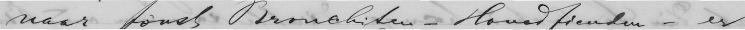naar først Bronchiten - Hovedfienden - er
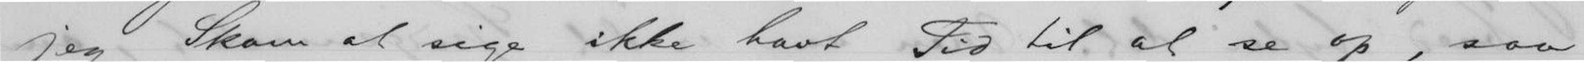jeg Skam at sige ikke havt Tid til at se op, saa
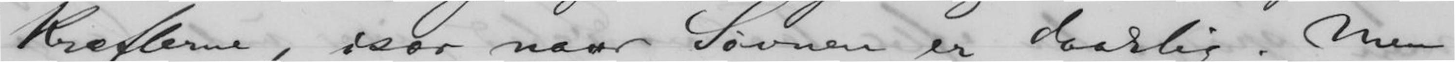Kræfterne, især naar Søvnen er daarlig. Men
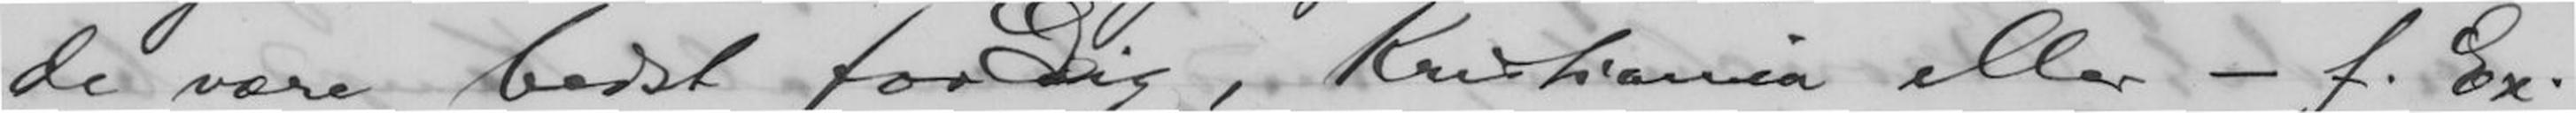de være bedst for Dig, Kristiania eller - f. Ex.
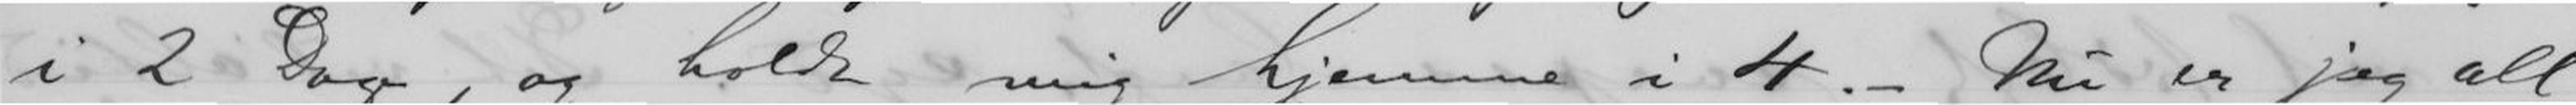i 2 Dage, og holdt mig hjemme i 4. - Nu er jeg all
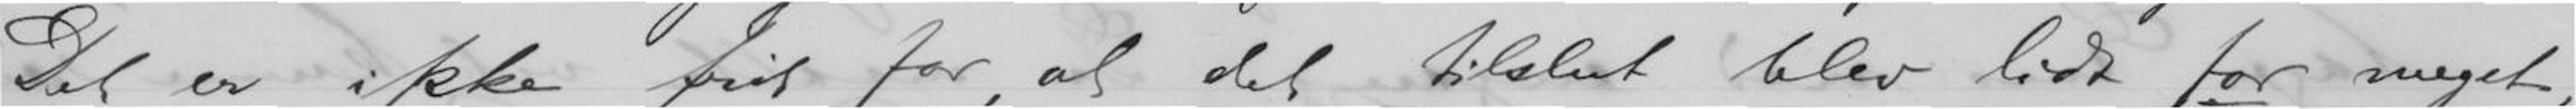Det er ikke frit for , at det tilslut blev lidt for meget,
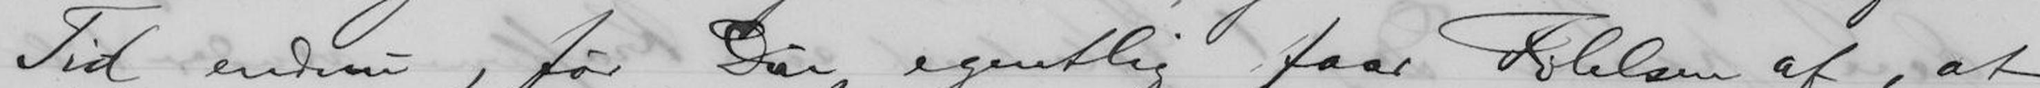Tid endnu, før Du egentlig faar Følelsen af, at
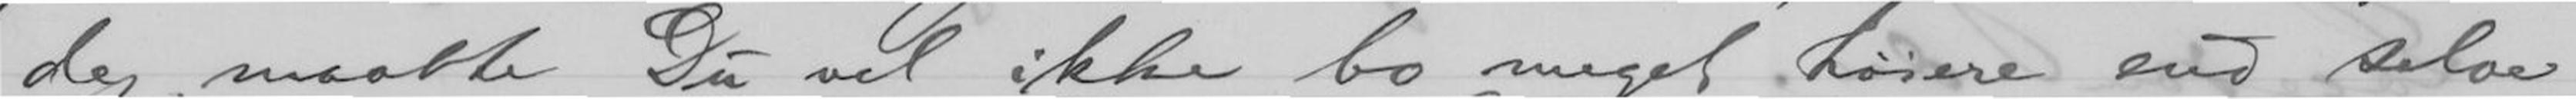de maatte Du vel ikke bo meget høiere end selve
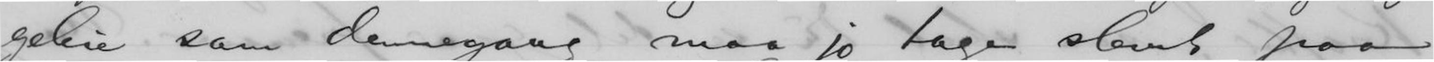geleie som dennegang maa jo tage slemt paa
